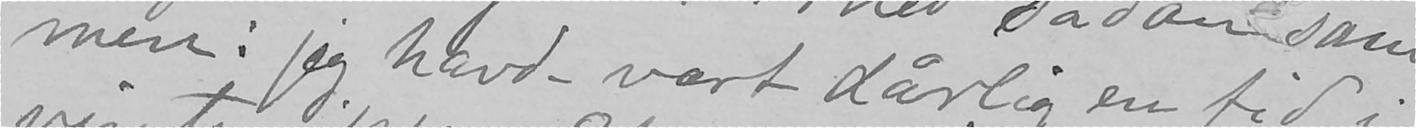men: jeg havde vært dårlig en tid i
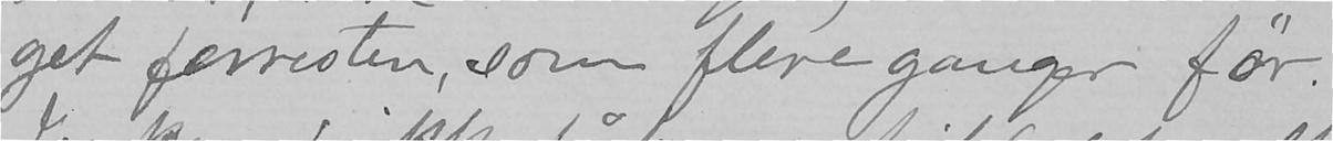get forresten, som flere ganger før.
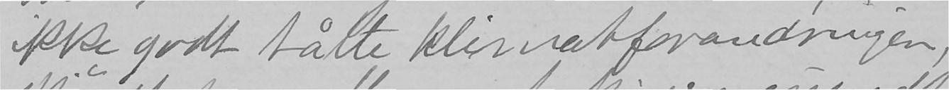ikke godt tålte klimatforandringen,
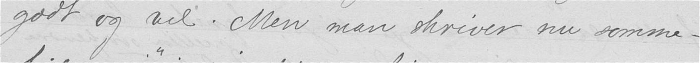godt og vel. Men man skriver nu somme-
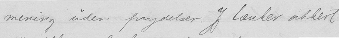mening uden prydelser. Jeg tænker sikkert
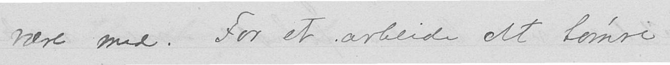være med. For et arbeide at tømre
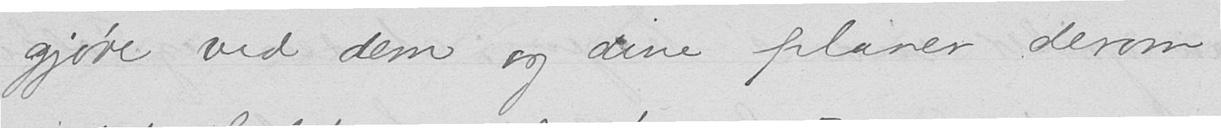gjøre ved dem og dine planer derom
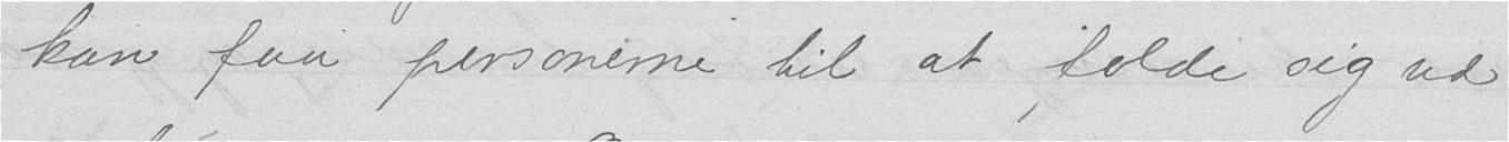kan faa personerne til at folde sig ud
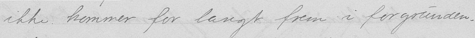ikke kommer for langt frem i forgrunden.
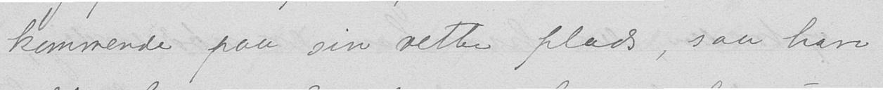kommende paa sin rette plads, saa han
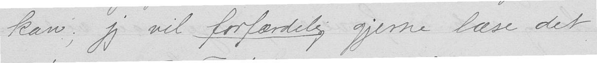kan; jeg vil forfærdelig gjerne læse det
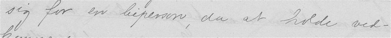sig for en biperson, da at holde ved-
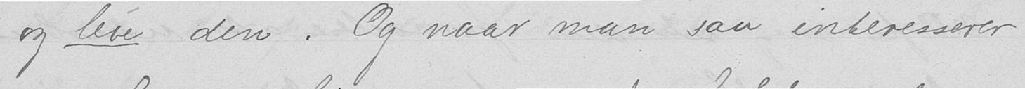og leve den. Og naar man saa interesserer
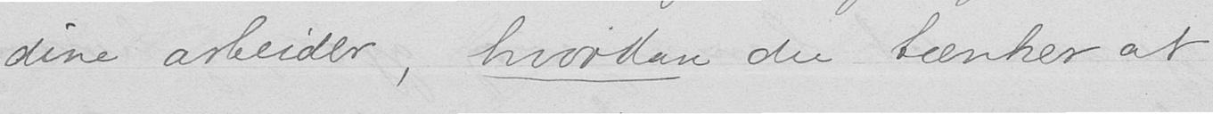dine arbeider, hvordan du tænker at
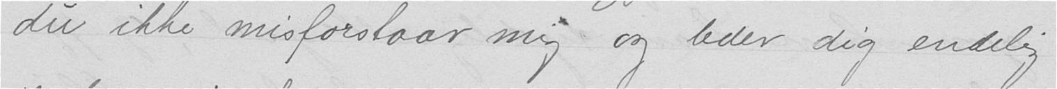du ikke misforstaar mig og beder dig endelig
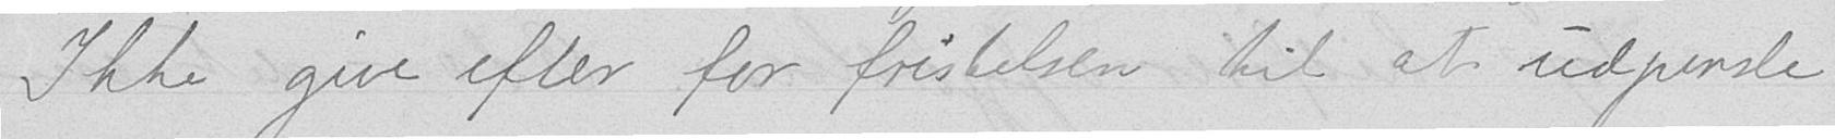Ikke give efter for fristelsen til at udpensle
